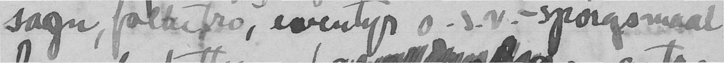sagn, folketro, eventyr o.s.v. - spørgsmaal
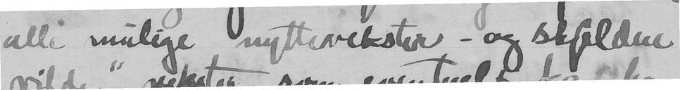alle mulige nyttevekster - og skjeldne
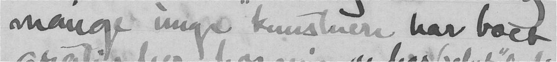mange unge kunstnere har boet
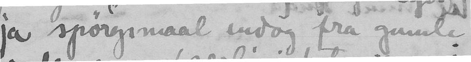ja spørgsmaal endog fra gamle
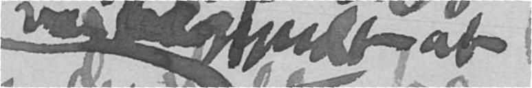var begyndt at
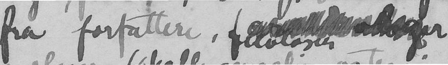fra forfattere,
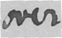over
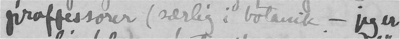proffessorer (særlig i botanik - jeg er
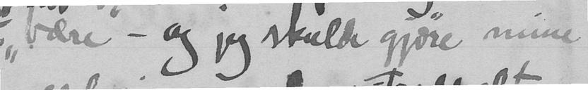"bære" - og jeg skulde gjøre mine
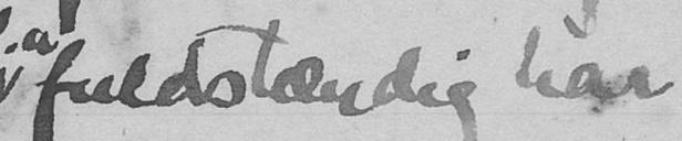fuldstændig har
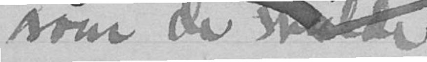som de
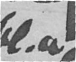bl. a
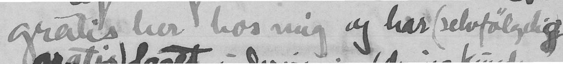gratis her hos mig og har (selvfølgelig
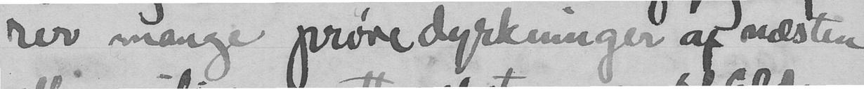rer mange prøvedyrkninger af næsten
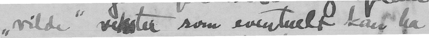"vilde" vekster som eventuelt kan ha
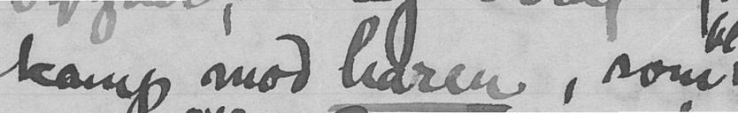kamp mod haren, som
